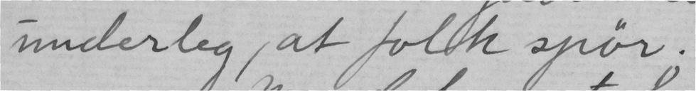underleg, at folk spør.
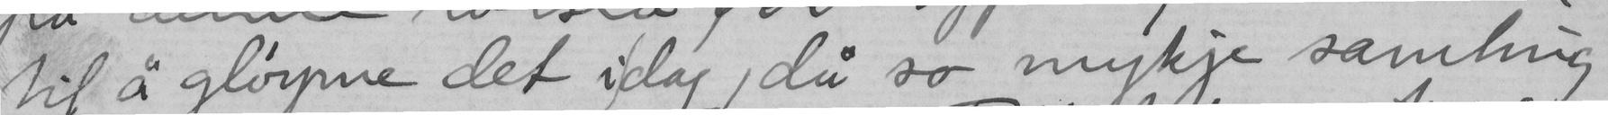til å gløyme det idag, då so mykje samling
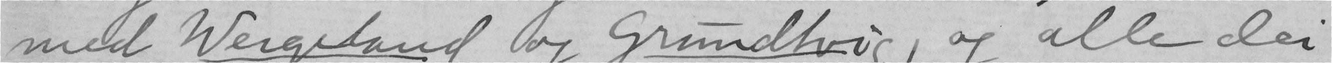med Wergeland og Grundtvig, og alle dei
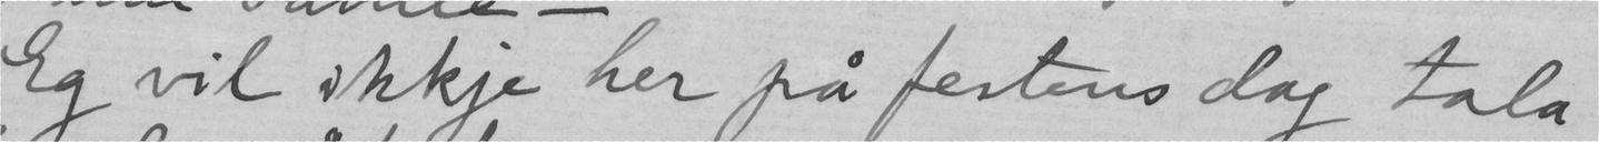Eg vil ikkje her på festens dag tala
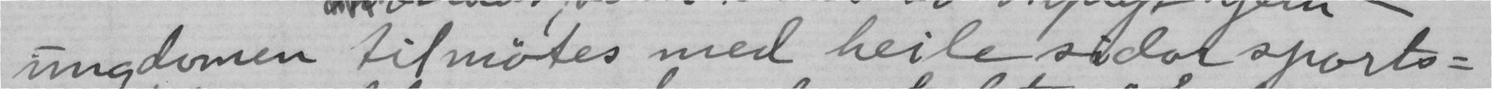ungdomen tilmøtes med heile sidar sports-
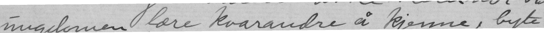ungdomen lære kvarandre å kjenne, byte
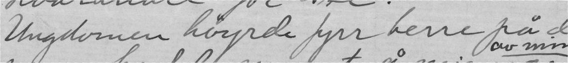Ungdomen høyrde fyrr berre på dei
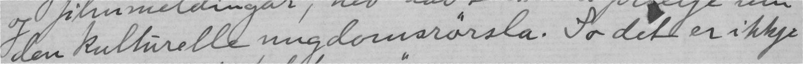den kulturelle ungdomsrørsla. So det er ikkje
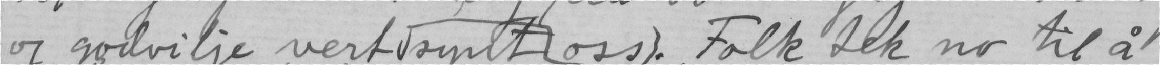og godvilje vert synt oss. Folk tek no til å
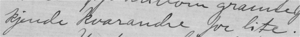kjende kvarandre for lite.
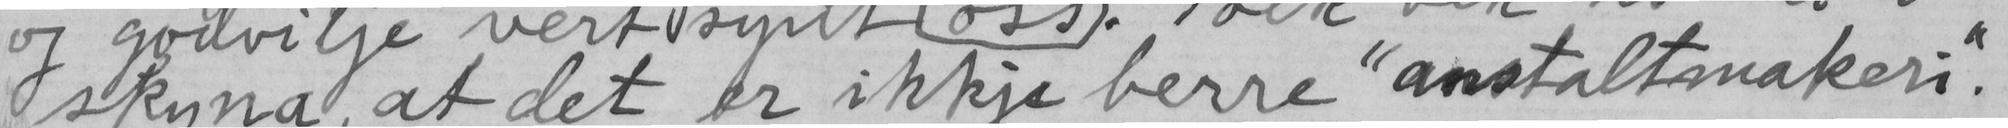skyna, at det er ikkje berre "anstaltmakeri".
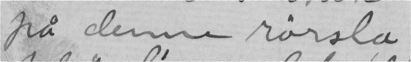på denne rørsla
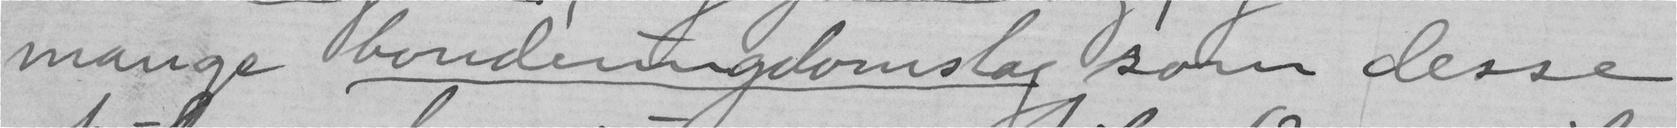mange bondeungdomslag som desse
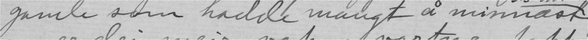gamle som hadde mangt å minnast
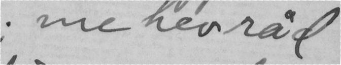me hev råd
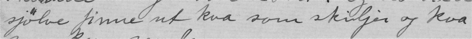sjølve finne ut kva som skiljer og kva
